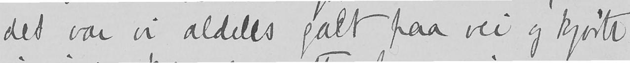det var vi aldeles galt paa vei og kjørte
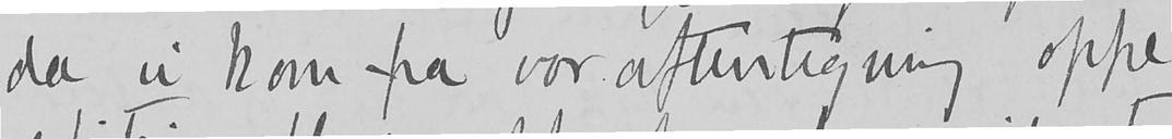da vi kom fra vor aftentegning oppe
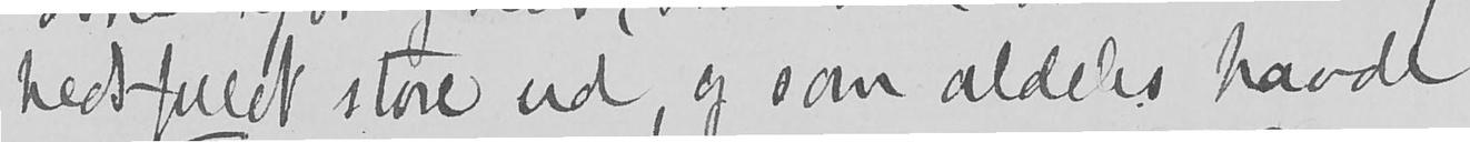hedsfuldt store ud, og som aldeles havde
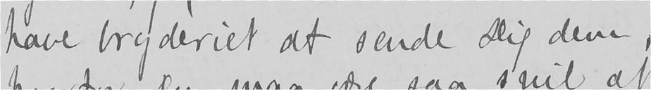have bryderiet at sende Dig dem,
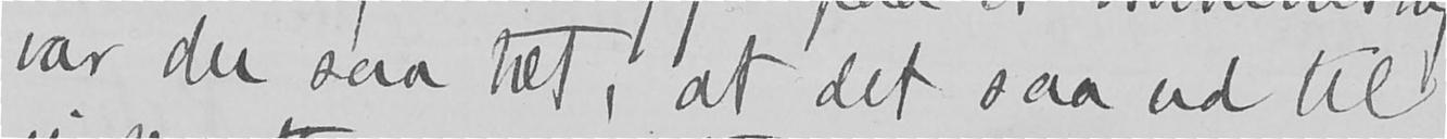var der saa tæt, at det saa ud til
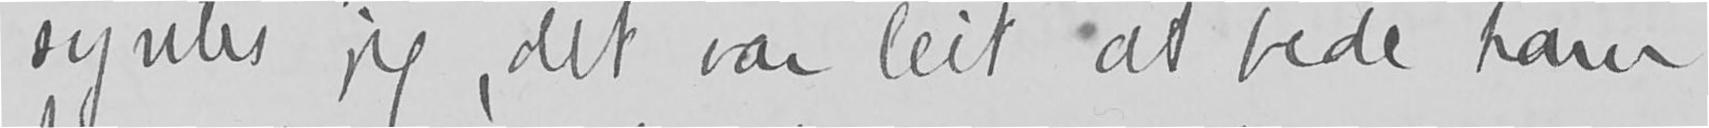syntes jeg, det var leit at bede ham
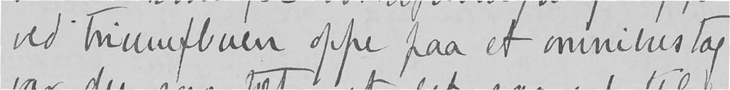ved Triumfbuen oppe paa et omnibustag
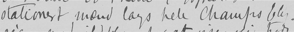stationert mænd lags hele Champs Ely-
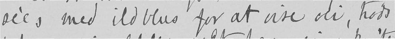sèes med ildblus for at vise vei, trods
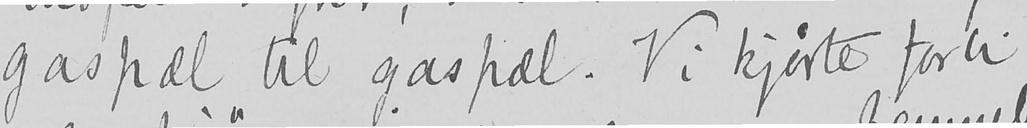gaspæl til gaspæl. Vi kjørte forbi
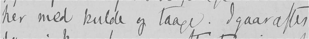her med kulde og taage. Igaaraftes
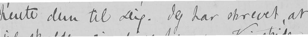hente dem til Dig. Jeg har skrevet, at
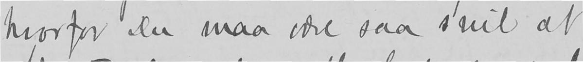hvorfor Du maa være saa snil at
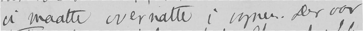vi maatte overnatte i vognen. Der var
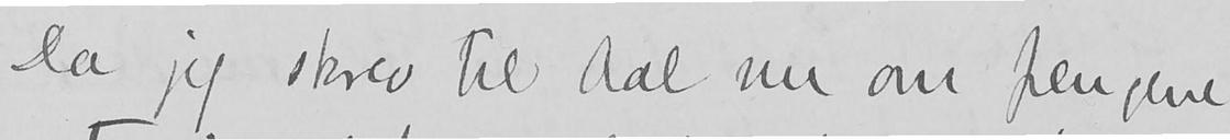Da jeg skrev til Aal nu om pengene
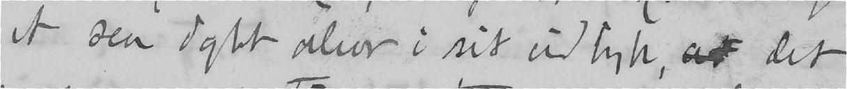et saa dybt alvor i sit udtryk, at det
